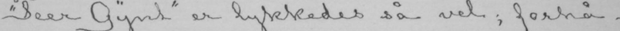«Peer Gynt» er lykkedes så vel; forhå-
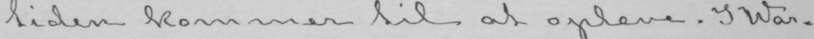tiden kommer til at opleve. I War-
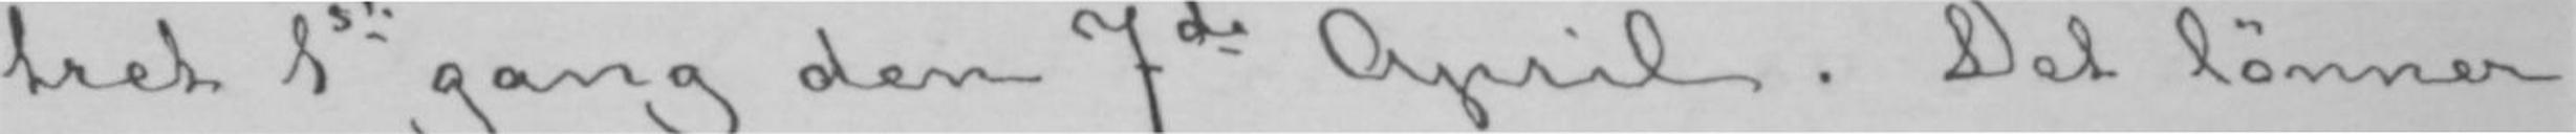tret 1ste gang den 7de April. Det lønner
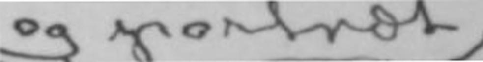og portræt;
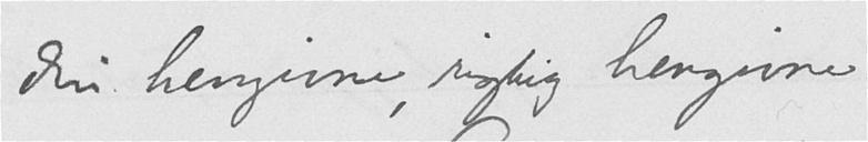din hengivne, rigtig hengivne
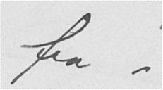fra
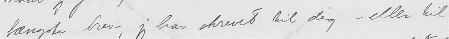længste brev, jeg har skrevet til dig - eller til
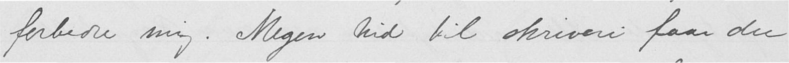forbedre mig. Megen tid til skriveri faar du
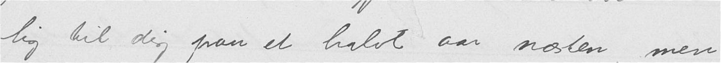lig til dig paa et halvt aar næsten, men
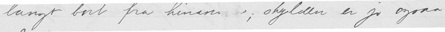langt bort fra hinanden; skylden er jo ogsaa
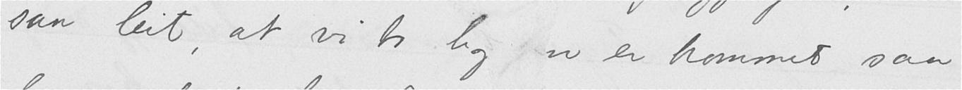saa leit, at vi to dog ligesom er kommet saa
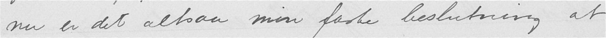nu er det altsaa min faste beslutning at
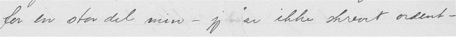for en stor del min - jeg har ikke skrevet ordent-
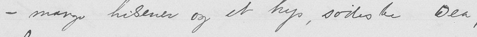- mange hilsener og et kys, sødeste Dea
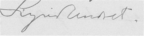Sigrid Undset.
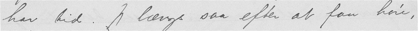har tid. Jeg længes saa efter at faa høre,
